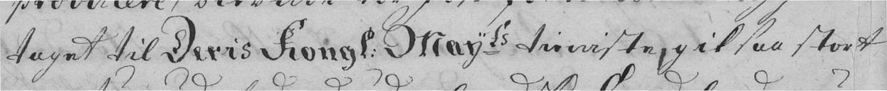taget til Deris Kongl. Mayt's tieniste, gik saa stort
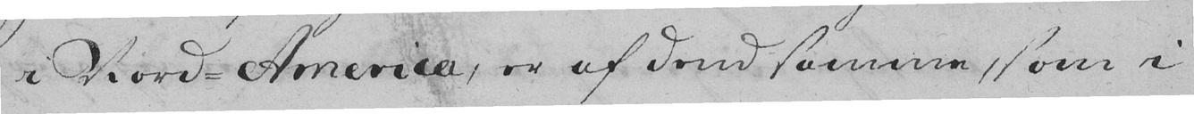i Nord-America, er af dend samme, som i
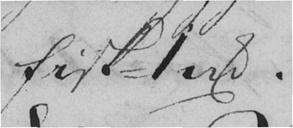fisk 1 mk.
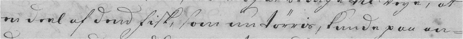en deel af dend Fisk, som nu tørris, kunde paa an-
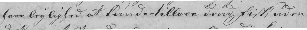have leylighed at kunde tillave dend Fisk, uden
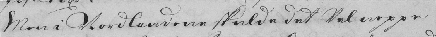Men i Nordlandene skulde det vel neppe
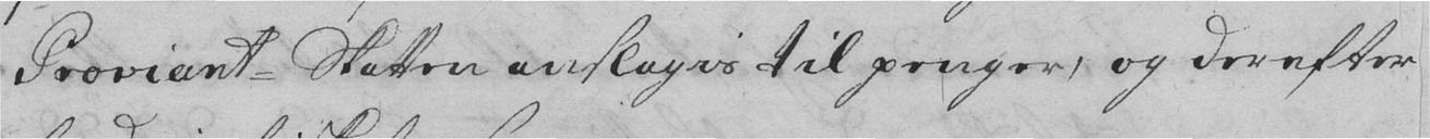Proviant-Skatten anslagis til penger, og derefter
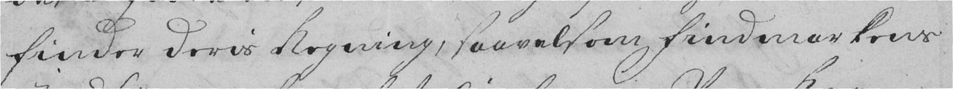finder deris Regning, saavelsom Findmarkens
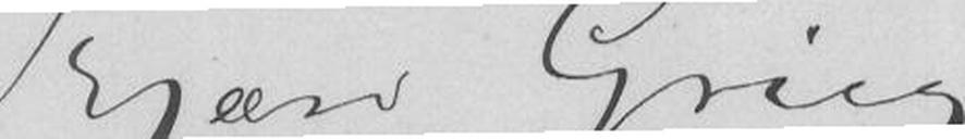Kjære Grieg
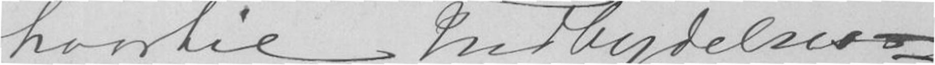hvortil Indbydelses-
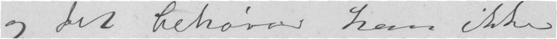og det behøver han ikke
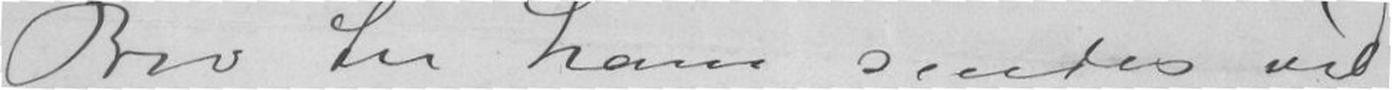Brev til ham sendes ved-
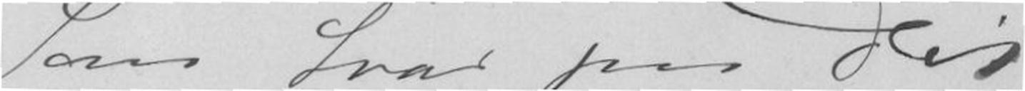Som Svar paa Dit
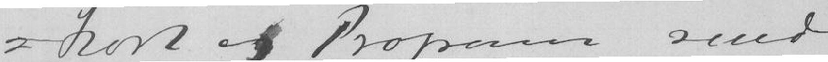kort og Program sendt
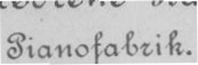Pianofabrik.
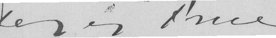Dig og Frue
